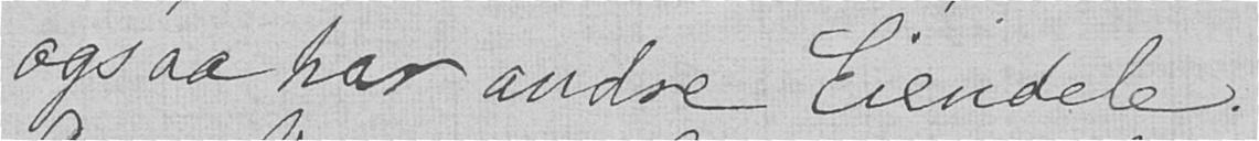ogsaa har andre Eiendele.
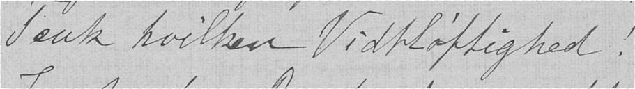Tenk hvilken Vidtløftighed!
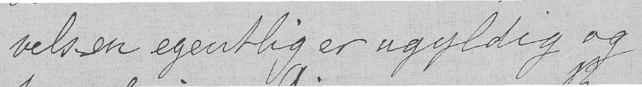velsen egentlig er ugyldig og
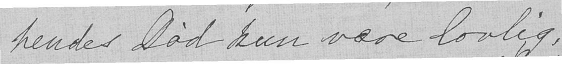hendes Død kun være lovlig,
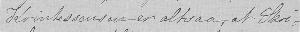Kvintessensen er altsaa, at Skri-
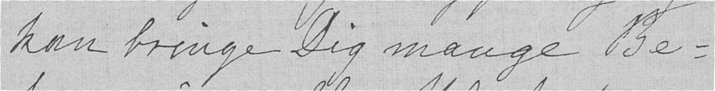kan bringe Dig mange Be-
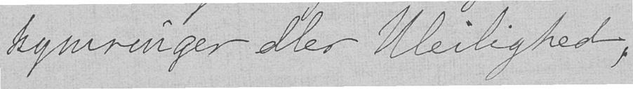kymringer eller Uleilighed,
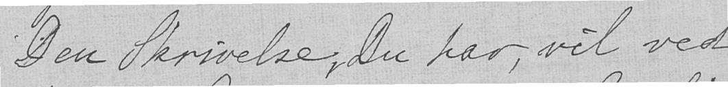Den Skrivelse du har, vil ved
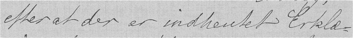efter at der er indhentet Erklæ-
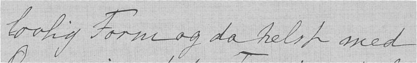lovlig Form og da helst med
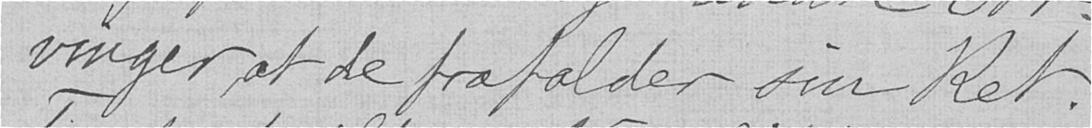vinger, at de frafalder sin Ret.
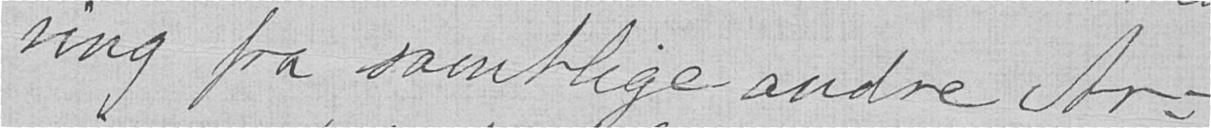ring fra samtlige andre Ar-
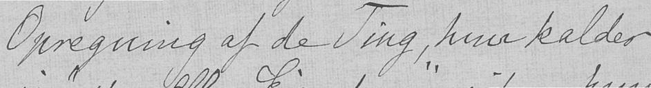Opregning af de Ting, hun kalder
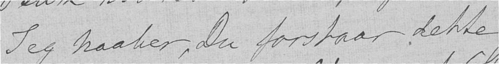Jeg haaber, Du forstaar dette.
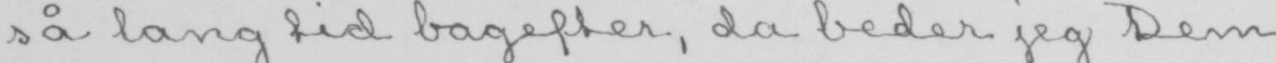så lang tid bagefter, da beder jeg Dem
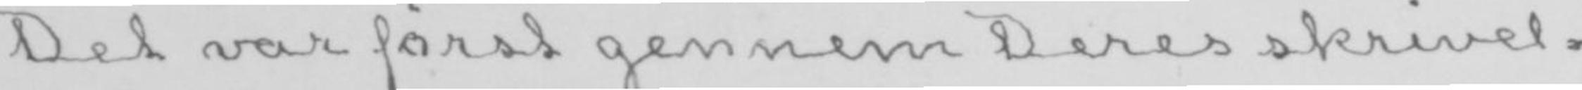Det var først gennem Deres skrivel-
